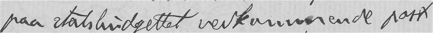paa statsbudgettet vedkommende post.
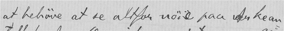at behøve at se altfor nøie paa Arkean-
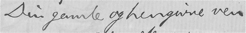Din gamle og hengivne ven
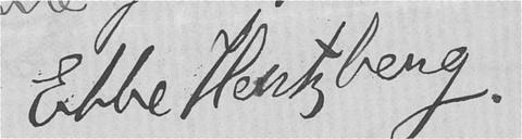Ebbe Hertzberg.
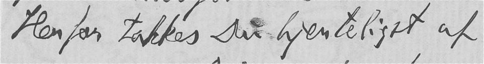Herfor takkes Du hjerteligst af
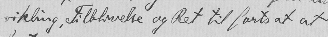vikling, Tilblivelse og Ret til fortsat at
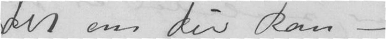det om du kan -
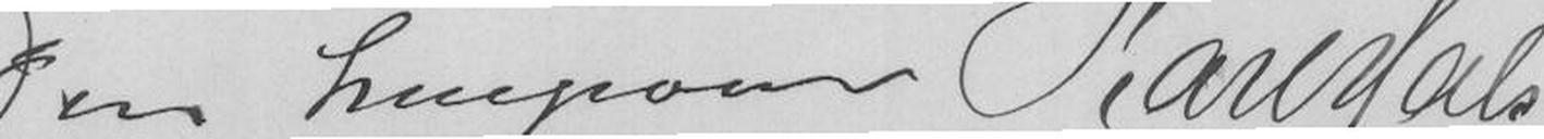Din hengivne Karl Hals
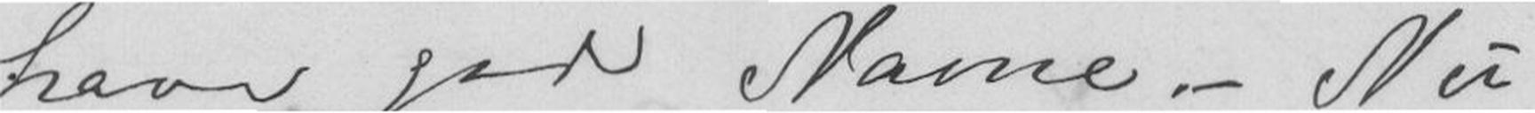have god Navne, - Nu
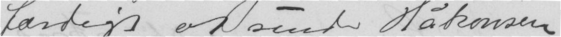færdigt at sende Håkonsen
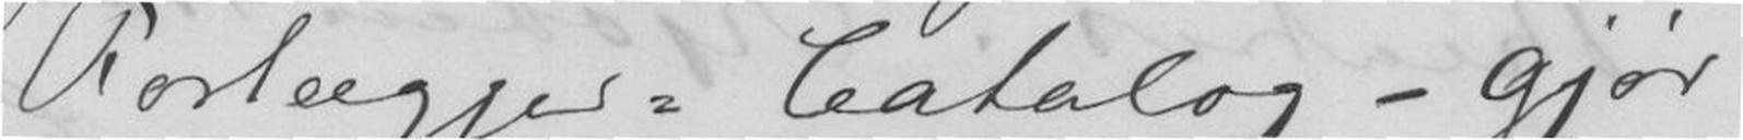Forlægger= Catalog - gjør
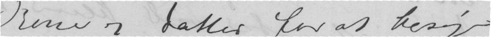Kone og Datter for at besøge
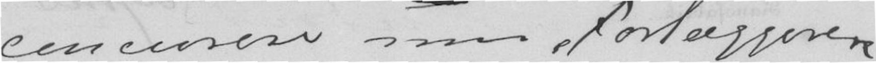concurere maa Forlæggeren
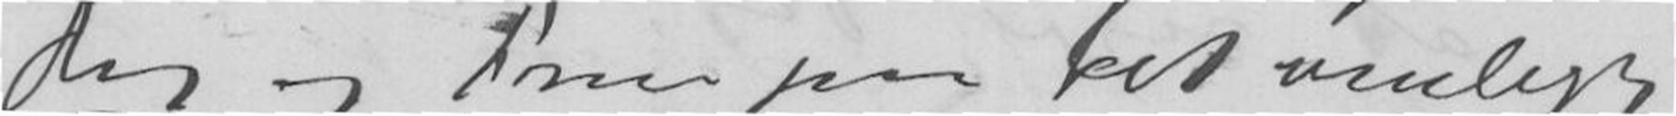Dig og Frue paa det venligste
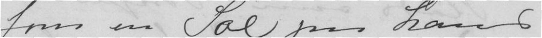som en Sol paa hans
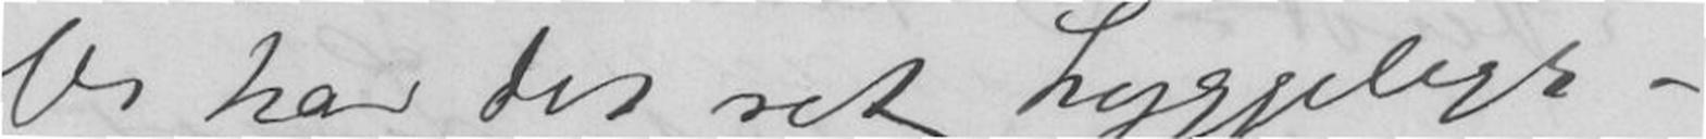Vi har det ret hyggeligt -
